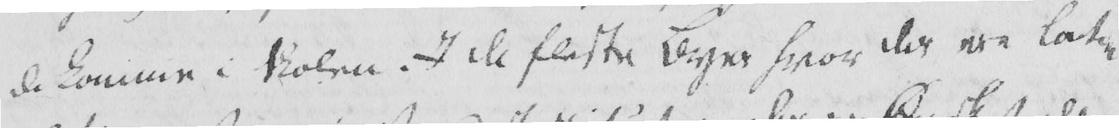de komme i Skolen. I de fleste Byer hvor der ere latin-
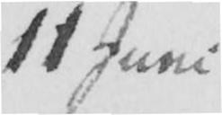11 Juni
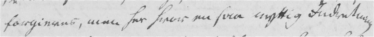forgieves, men her hvor en saa nyttig Indretning
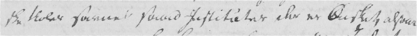ske Skoler savnes saad Instituter der er Ønsket altsaa
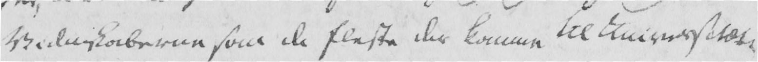Videnskaberne som de fleste der komme til Universitætet
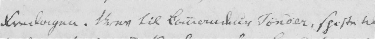Fredagen. Skrev til Komandeur Tønder, spiste til
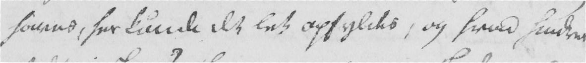haves, her kunde det let opfyldes, og hvad hindrer
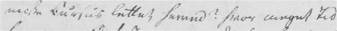miske Cursus lettet herved? Hvor meget tid
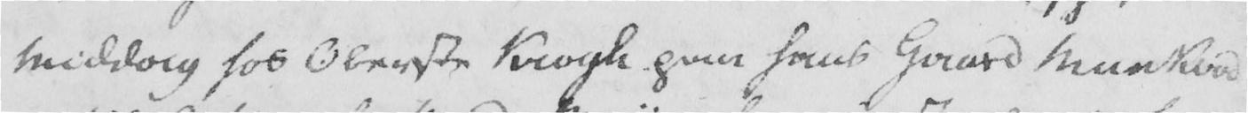Middag hos Oberste Krogh paa hans Gaard Munkvold
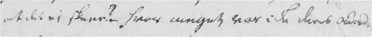at det ei skeer? hvor meget var icke deres acade-
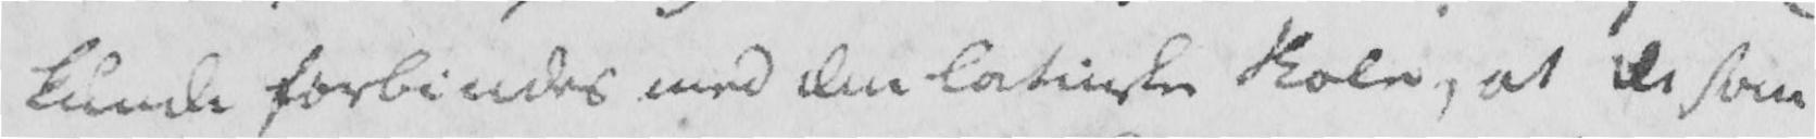kunde forbindes med den latinske Skole, at de som
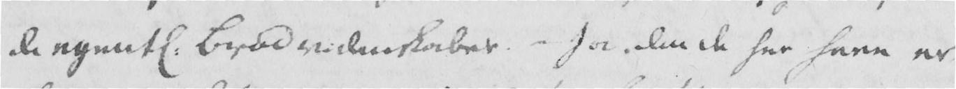de egentl. Brødvidenskaber. - Ja den de her have er
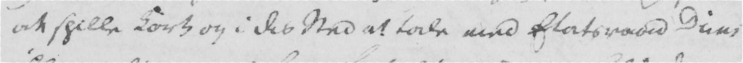at spille Kort og i des Sted at tale med Etatsraad Duns
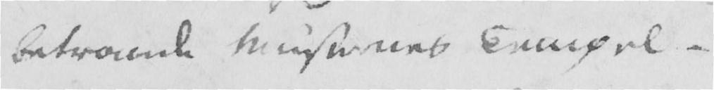betraaede Muserne Tempel. -
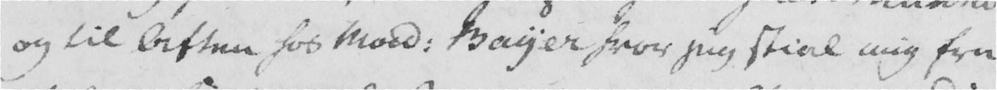og til Aften hos Mad. Bayer hvor jeg stial mig fra
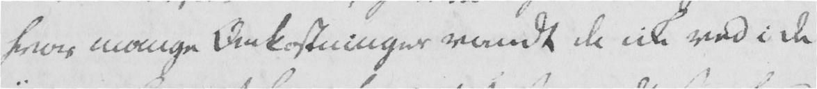hvor mange Omkostninger vandt de icke ved i de
